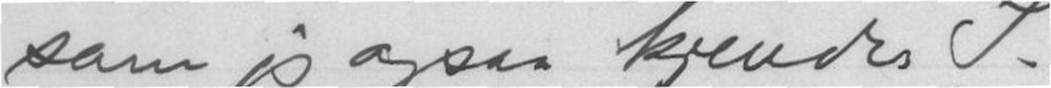som jeg ogsaa kjendes S.
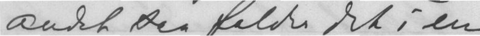andet saa falder det i en
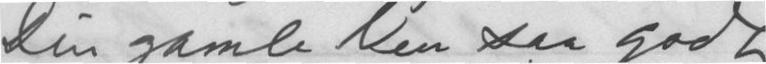Din gamle Ven saa godt,
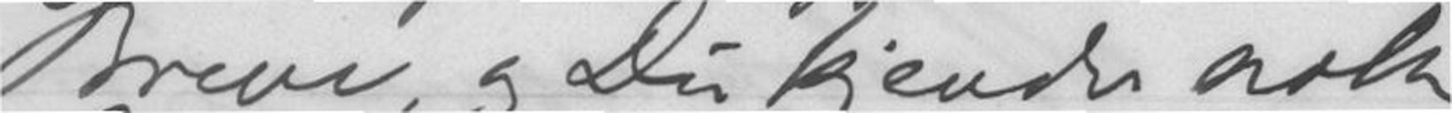Breve, og Du kjender nok
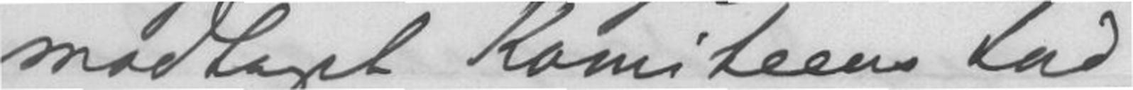modtaget Komiteens Ind-
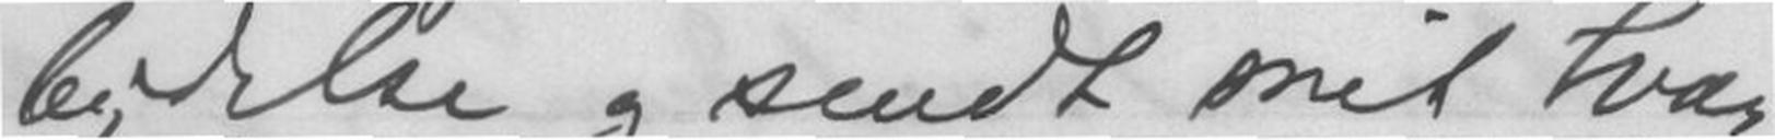bydelse og sendt mit Svar
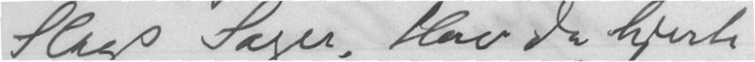Slags Sager. Hav da hjerte-
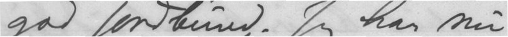god jordbund. Jeg har nu
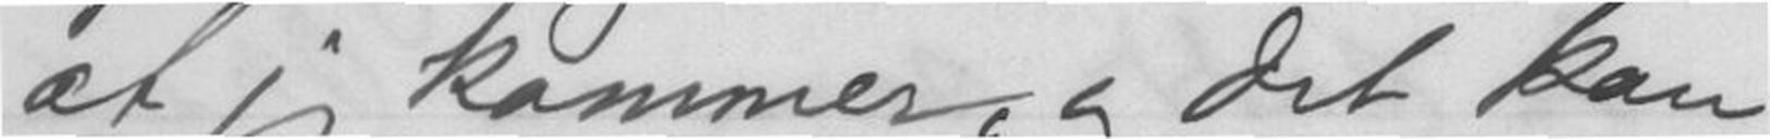at jeg kommer, og det kan
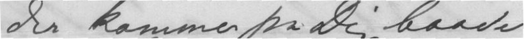der kommer fra Dig baade
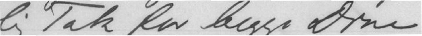lig Tak for begge Disse
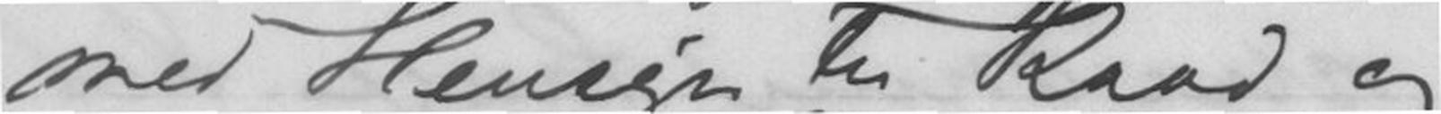med Hensyn til Raad og
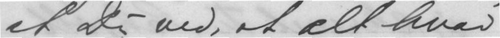at Du ved, at alt hvad
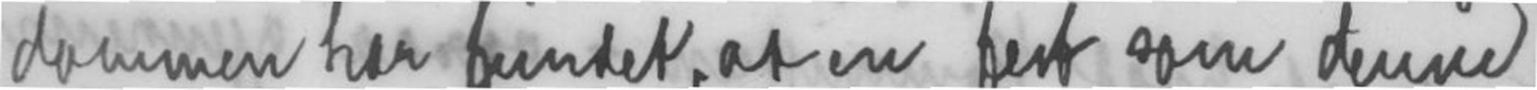dommen har fundet, at en fest som denne
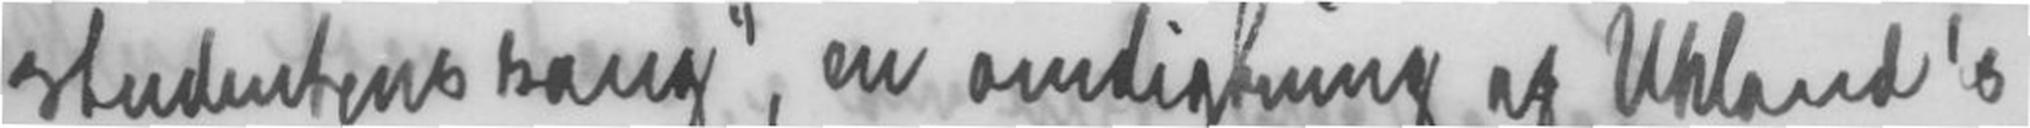studentens sang, en omdigtning af Uhland's
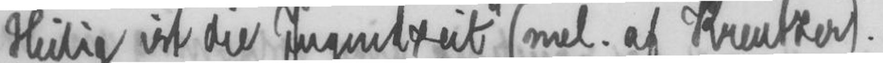Heilig ist die Jugendreit (mel. af Kreutzer).
